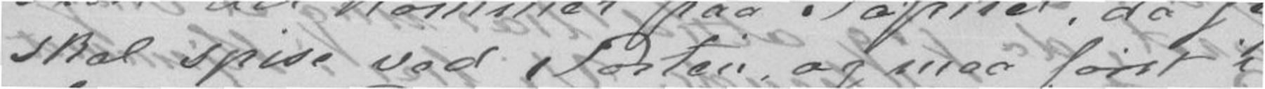skal spise ved Posten, og maa først i
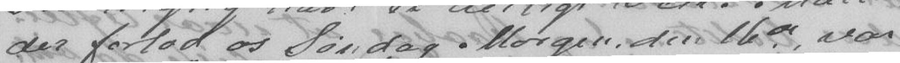der forlod os Søndag Morgen den 16de, var
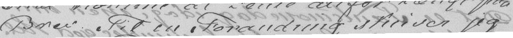Brev. Til en Forandring skriver jeg
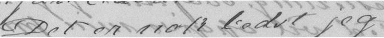Det er nok bedst jeg
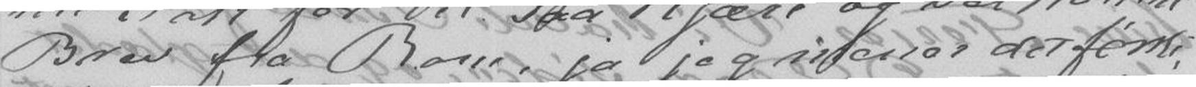Brev fra Rom, ja jeg mener det første,
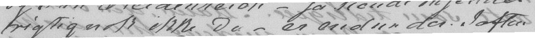rigtignok ikke Du - er endnu der. Iaften
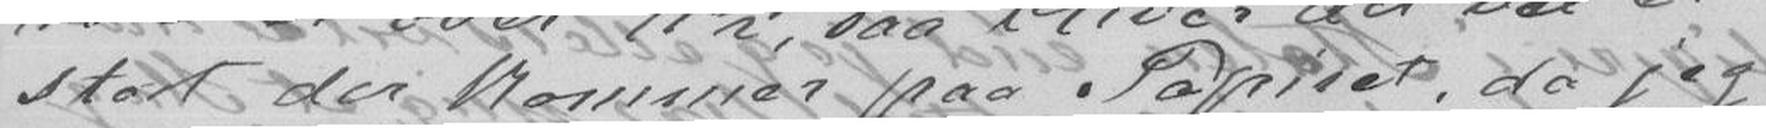stort der kommer paa Papiret, da jeg
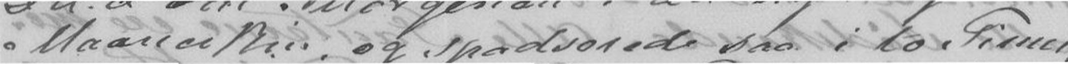Maaneskin, og spadserede saa i to Timer,
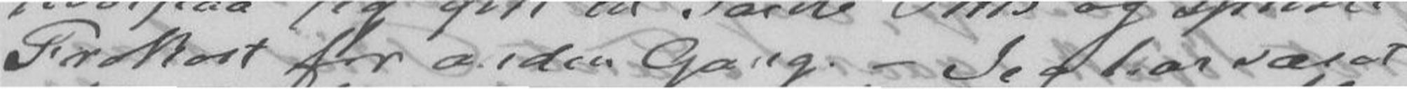Frokost for anden Gang. - Jeg har været
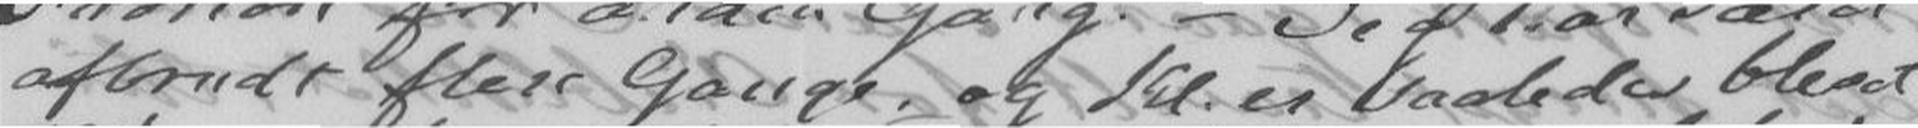afbrudt flere Gange, og Kl. er saaledes blevet
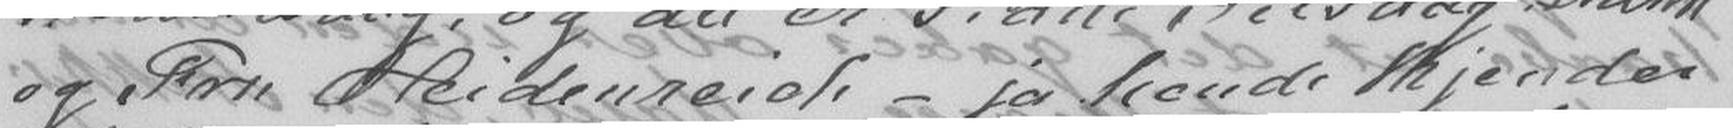og Fru Heidenreich - ja hende kjender
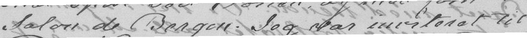Salon de Bergen. Jeg var inviteret til
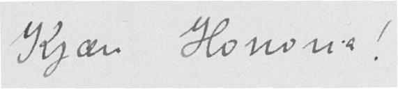Kjære Honoria!
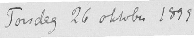Torsdag 26 oktober 1899
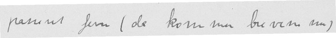passert fem (de kom med brevene nu)
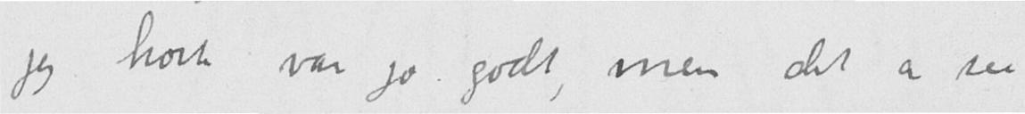jeg hørte var jo godt, men det er saa
